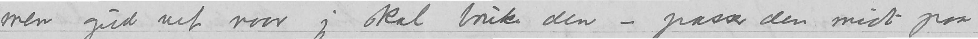men gud vet naar jeg skal bruke den – passer den midt paa
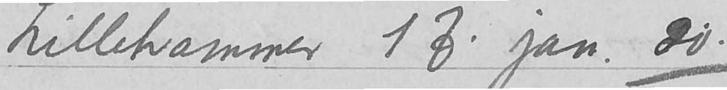Lillehammer 17. jan. 20.
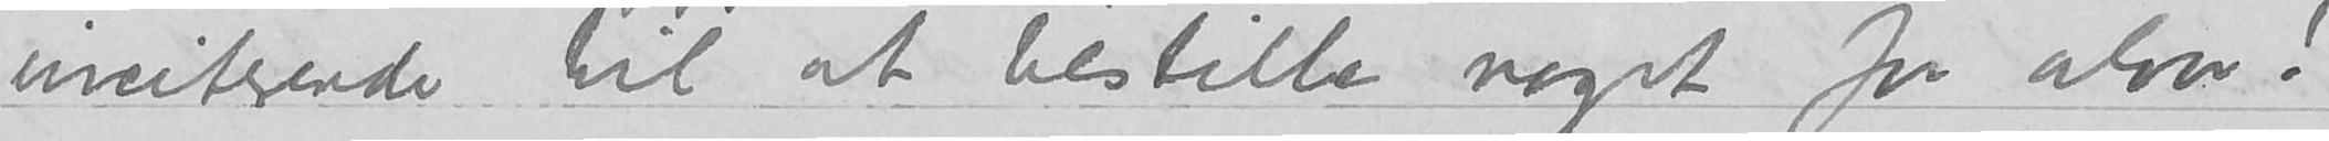inciterende til at bestille noget for alvor!
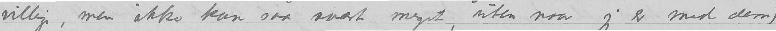flinke og villige, men ikke kan saa svært meget, uten naar jeg er med dem.)
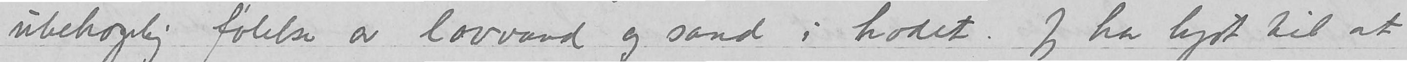ubehagelig følelse av lavvand og sand i hodet.  Jeg har lyst til at
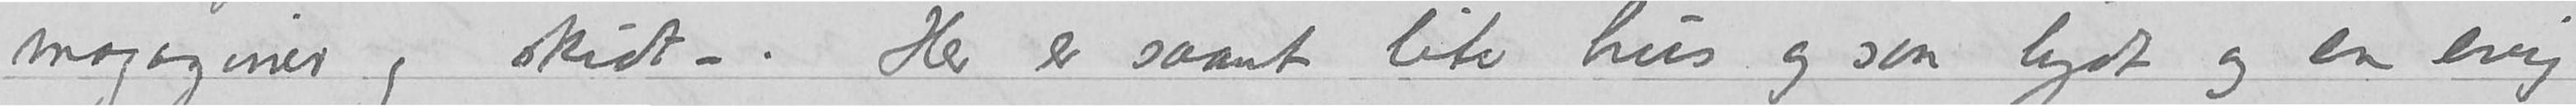magaziner og skidt –.  Her er saant lite hus og saa lydt og en evig
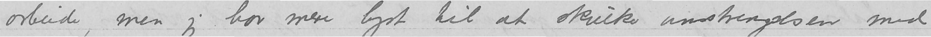arbeide, men jeg har mere lyst til at skulke anstrengelsen med
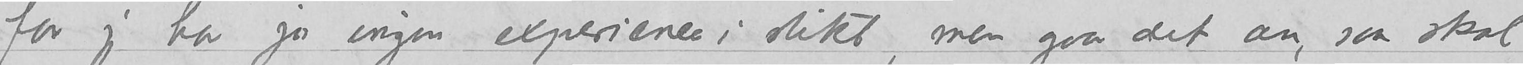for jeg har jo ingen experience i slikt, men gaar det an, saa skal
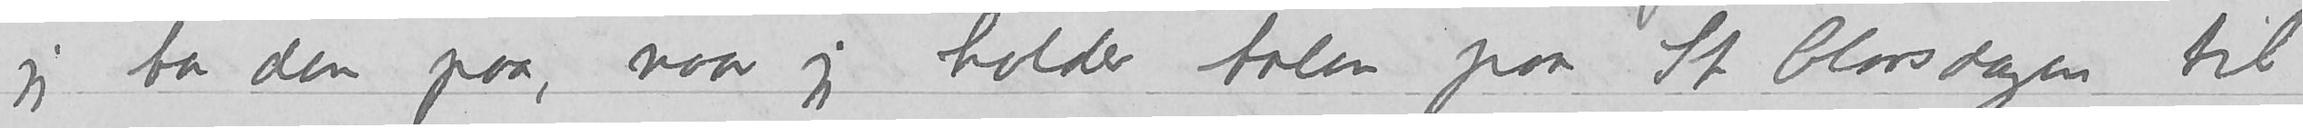jeg ta den paa, naaar jeg holder talen paa St. Olavsdagen til
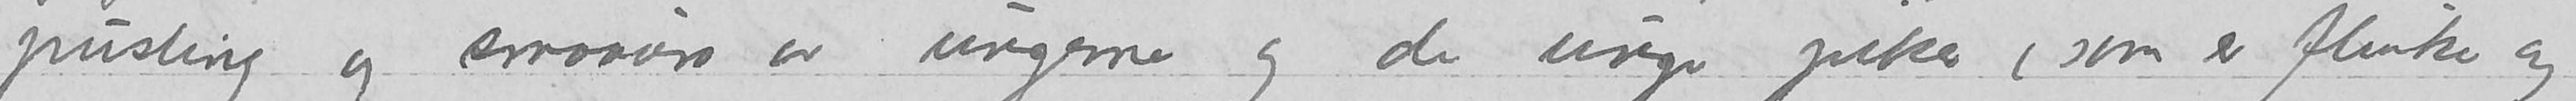pusling og smaauro av ungerne og de unge piker (som er
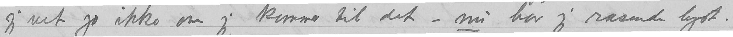jeg vet jo ikke om jeg kommer til det – nu har jeg rasende lyst.
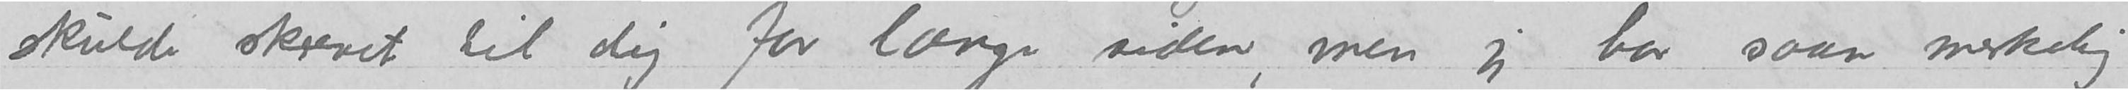skulde skrevet til dig for længe siden, men jeg har saan merkelig
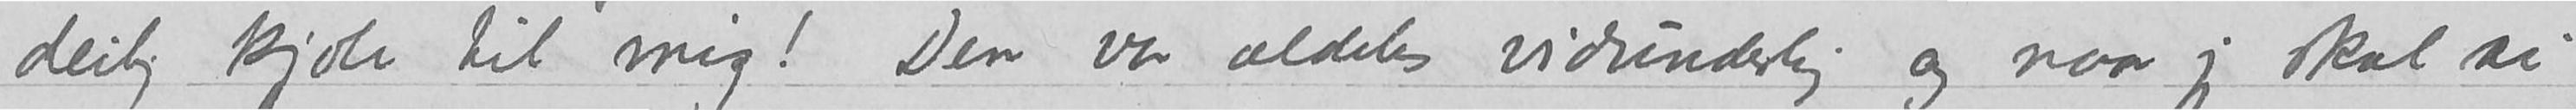deilig kjole til mig! Den var aldeles vidunderlig og naar jeg skal si
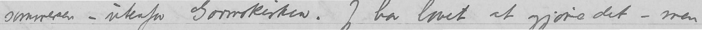sommeren – utenfor Garmokirken.  Jeg har lovet at gjøre det – men
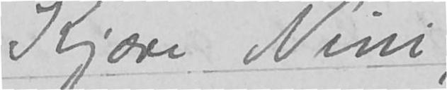Kjære Nini
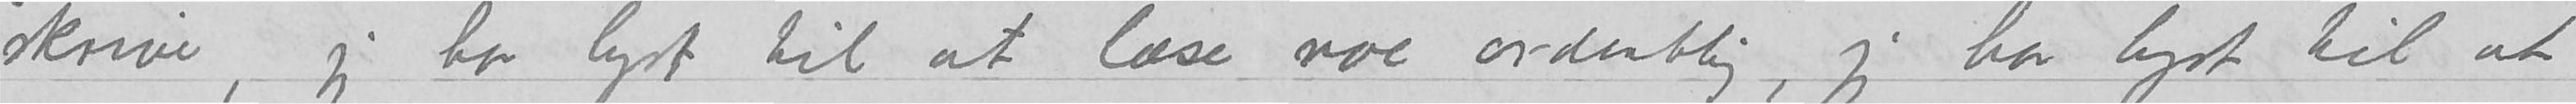skrive, jeg har lyst til at læse noe ordentlig, jeg har lyst til at
